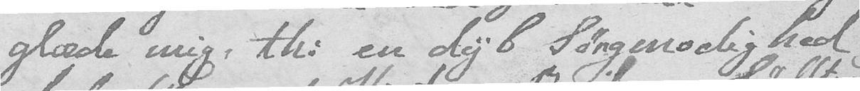glæde mig, thi en dyb Sørgmodighed
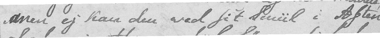men ej kan den ved sit Smiil i Aften
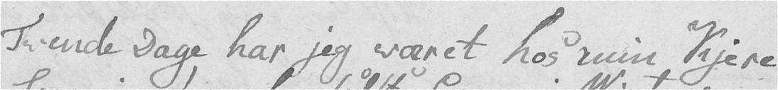Tvende Dage har jeg været hos min Kjere
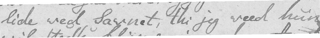lide ved Savnet, thi jeg veed hun
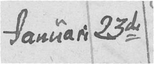Januari 23de
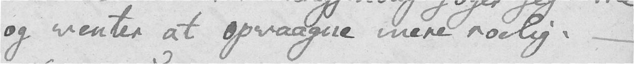og venter at opvaagne mere roelig. –
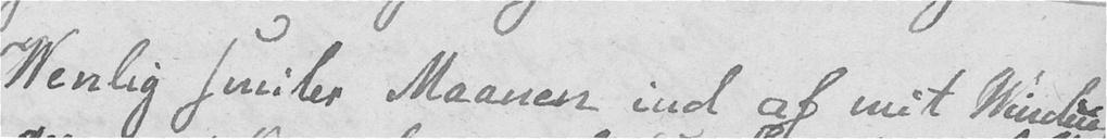Venlig smiler Maanen ind af mit Vindue,
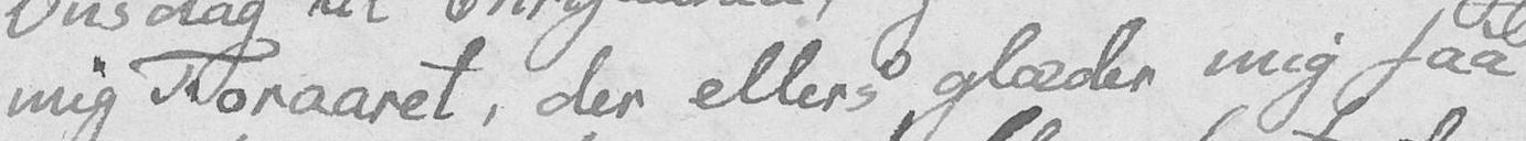mig Foraaret, der ellers glæder mig saa
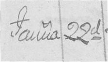Janua 22d
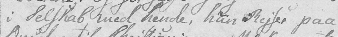i Selskab med hende, hun Rejser paa
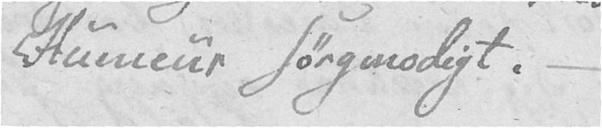Humeur sørgmodigt. –
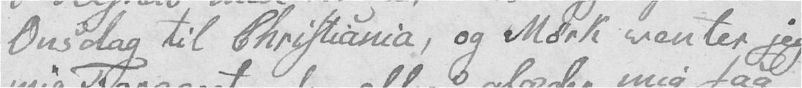Onsdag til Christiania, og Mørk venter jeg
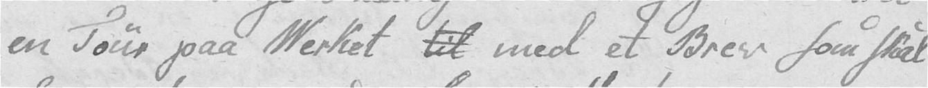en Tour paa Verket til med et Brev som skal
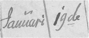Januari 19de
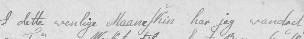I dette venlige Maaneskin, har jeg vandret
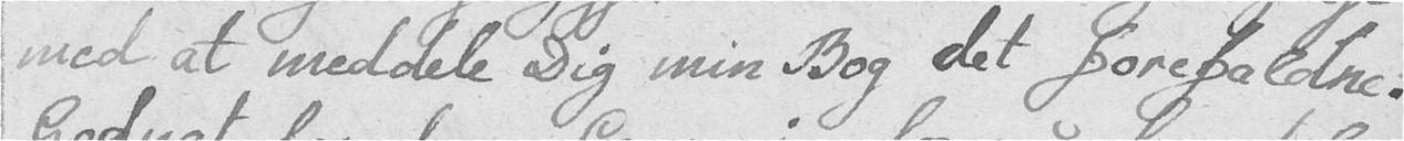med at meddele Dig min Bog det forefaldne.
